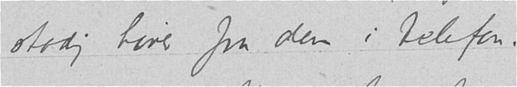stadig hører fra dem i telefon.
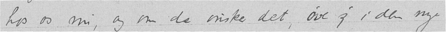hos os nu, og om de ønsker det, øve sig i den nye
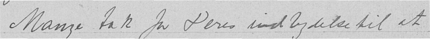Mange tak for Deres indsbydelse til at
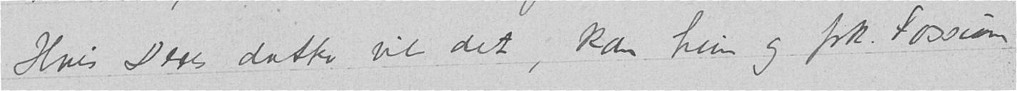Hvis Deres datter vil det, kan hun og frk. Fossum
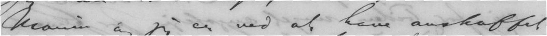Marie og jeg er ved at have anskaffet
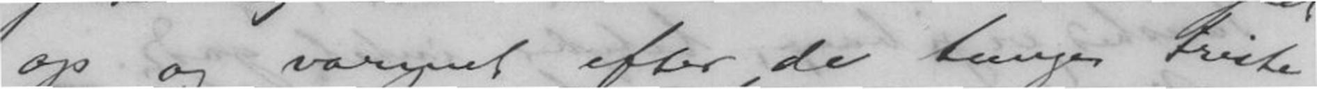op og varmet efter de tunge triste
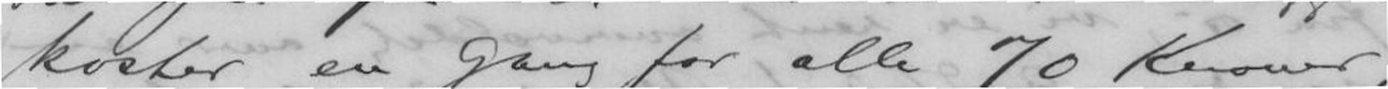koster en Gang for alle 70 Kroner,
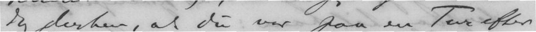dog derhen, at du var paa en Tur efter
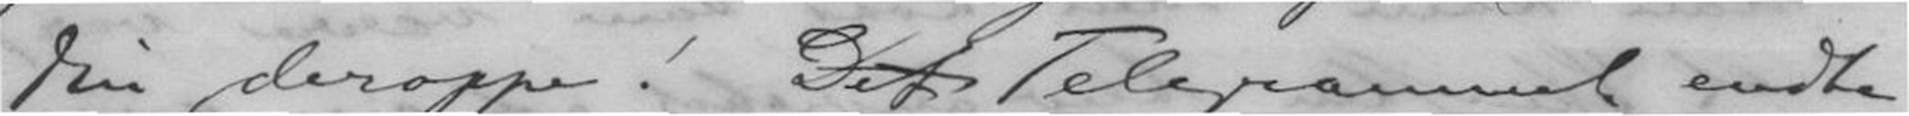din deroppe! Det Telegrammet endte
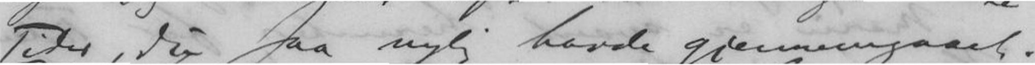Tider, du saa nylig havde gjennemgaaet.
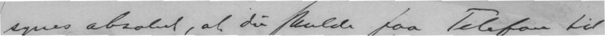synes absolut, at du skulde faa Telefon til
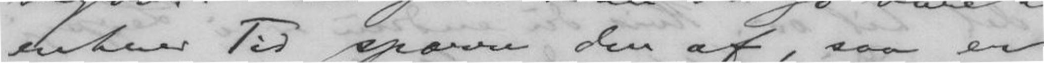enhver Tid spærre den af, saa er
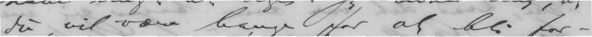du vil være bange for at bli for-
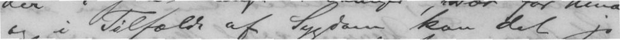og i Tilfælde af Sygdom kan det jo
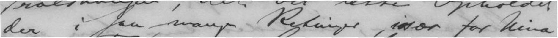der i saa mange Retninger, især for Nina,
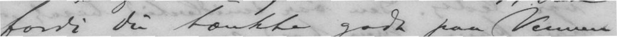fordi du tænkte godt paa Vennen
